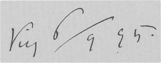Viig 6/9 95.
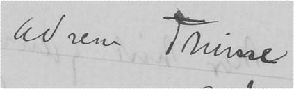ad sen Thime
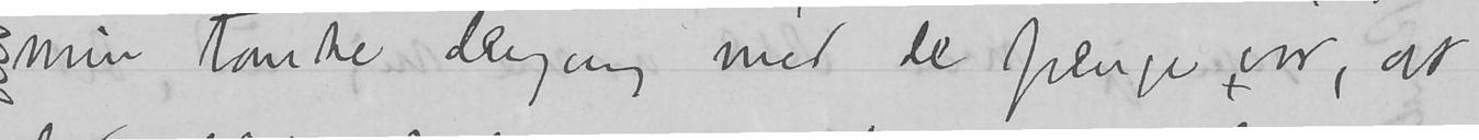min tanke dengang med de penger, var, at
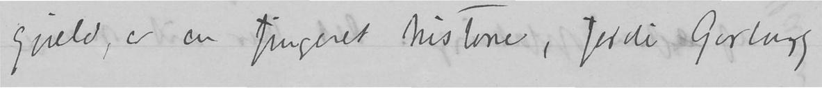gjæld, er en fingeret histore, fordi Garborg
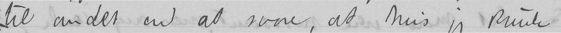til andet end at svare, at hvis jeg kunde
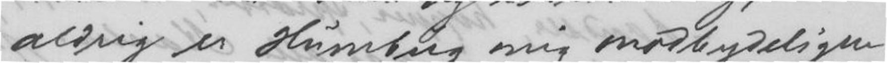aldrig er Humbug mig modbydeligere
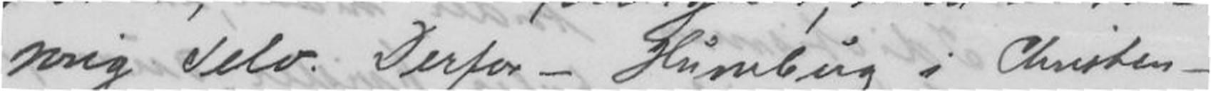mig selv. Derfor - Humbug i Christen-
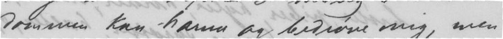dommen kan harme og bedrøve mig, men
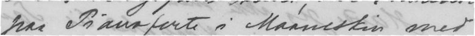paa Pianoforte i Maaneskin med
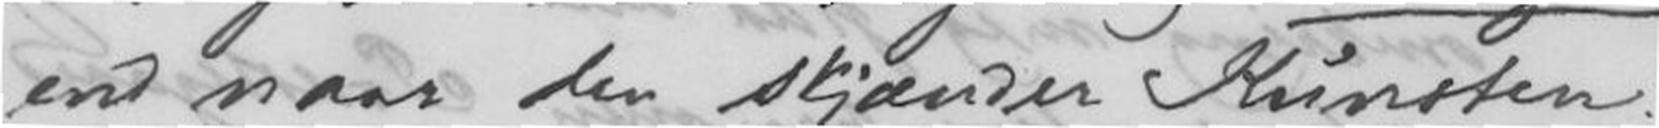end naar den skjænder Kunsten.
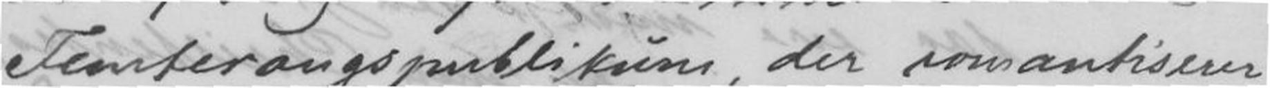Femterangspublikum, der romantiserer
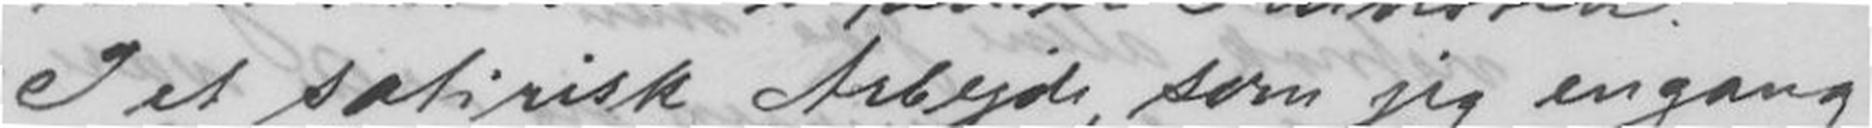I et satirisk Arbejde, som jeg engang
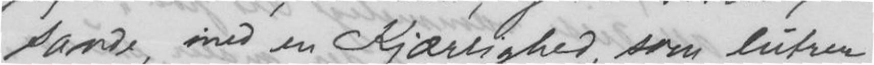sande, med en Kjærlighed, som lutrer
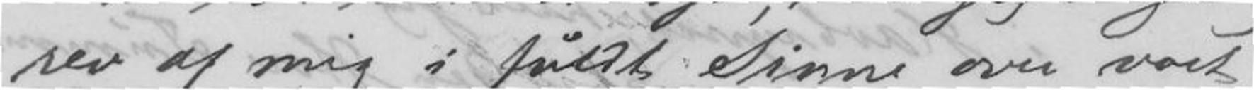rev af mig i fuldt sinne over vort
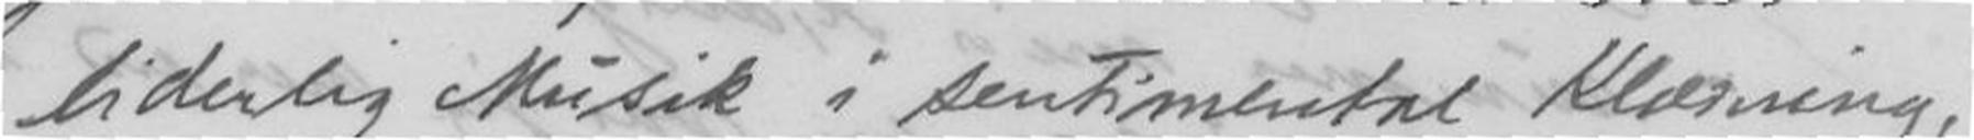liderlig Musik i sentimental Klædning,
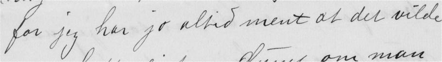for jeg har jo altid ment at det vilde
